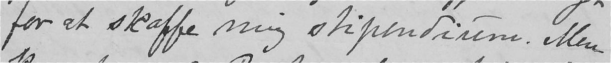for at skaffe mig stipendium. Men
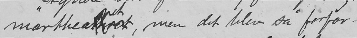martheatret, men det blev så forfær-
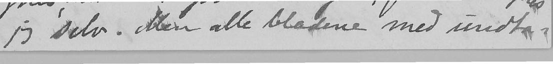jeg selv. Men alle bladene med undta-
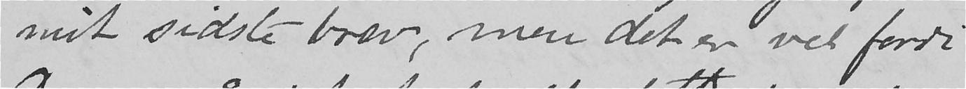mit sidste brev, men det er vel fordi
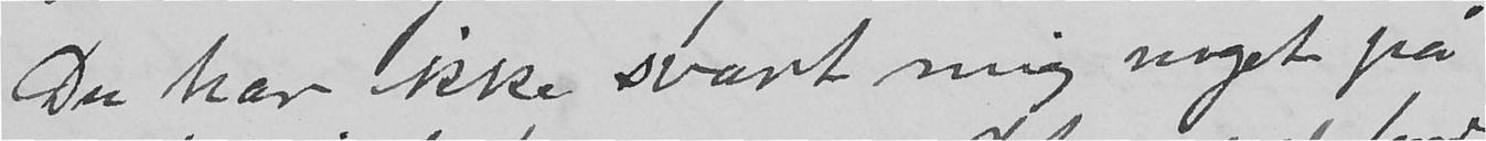Du har ikke svart mig noget på
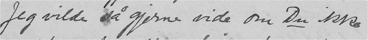Jeg vilde så gjerne vide om Du ikke
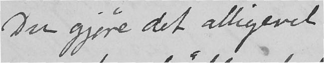Du gjøre det alligevel
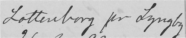Lottenborg pr. Lyngby
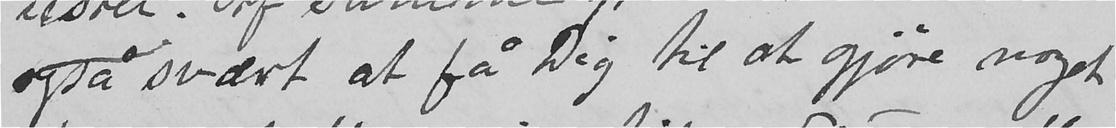også svært at få Dig til at gjøre noget
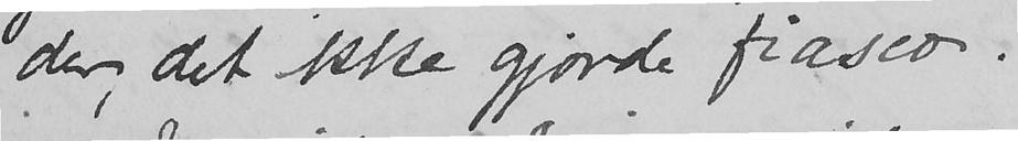der det ikke gjorde fiasco.
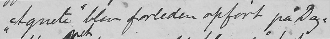"Agnete" blev forleden opført på Dag-
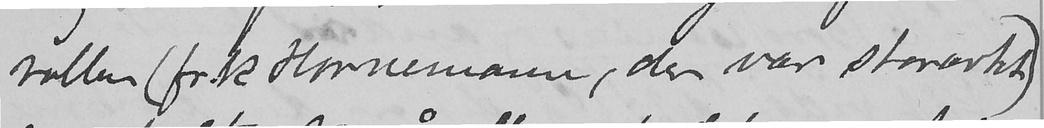rollen (frk Hornemann, der var storartet)
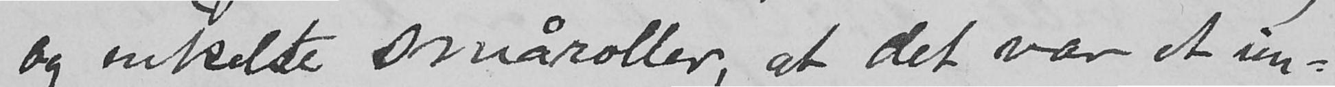og enkelte småroller, at det var et un-
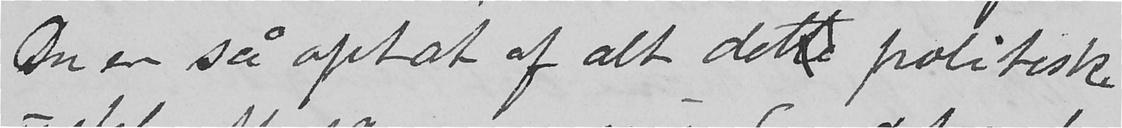Du er så optat af alt dette politiske
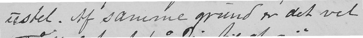ustel. Af samme grund er det vel
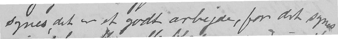synes, det er et godt arbejde, for det synes
